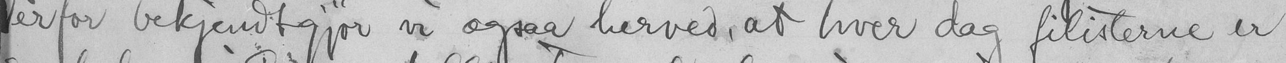Derfor bekjendtgjør vi ogsaa herved, at hver dag filisterne er
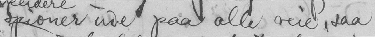ude paa alle veie, saa
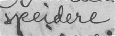speidere
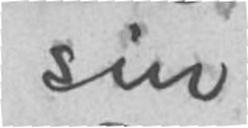sin
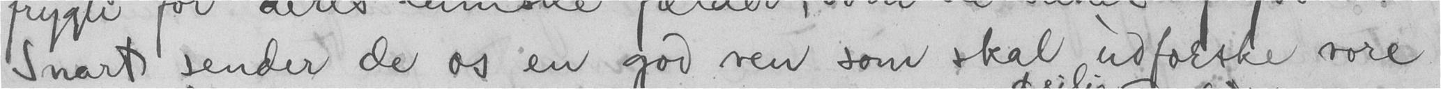Snart sender de os en god ven som skal udforske vore
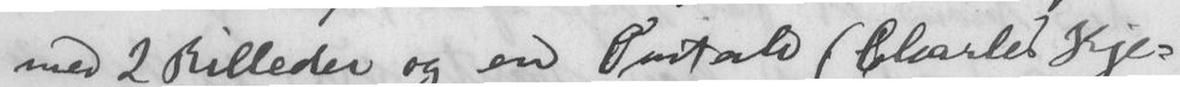med 2 Billeder og en Omtale (Charles Kje-
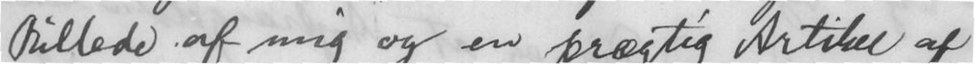Billede af mig og en prægtig Artikel af
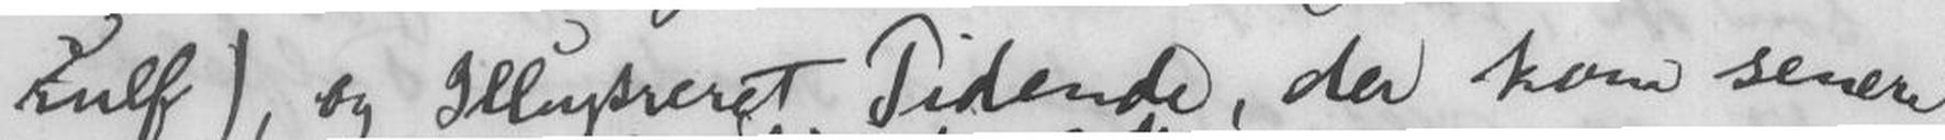kulf), og Illustreret Tidende, der kom senere
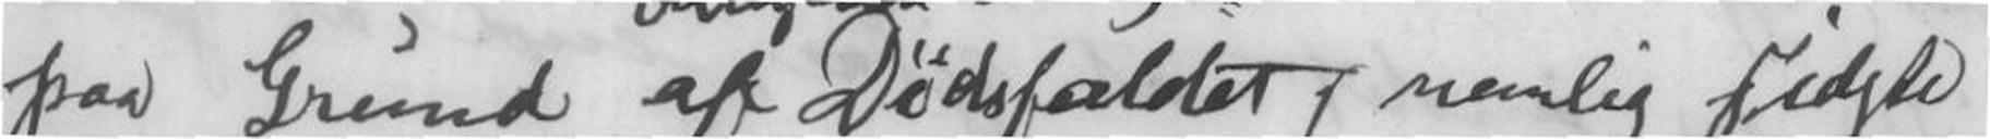paa Grund af Dødsfaldet, nemlig sidste
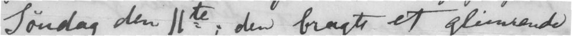Søndag den 11te; den bragte et glimrende
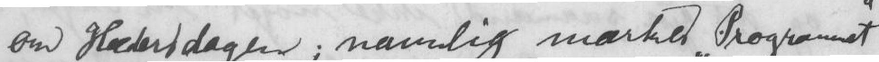om Hædersdagen; navnlig mærkes Programmet
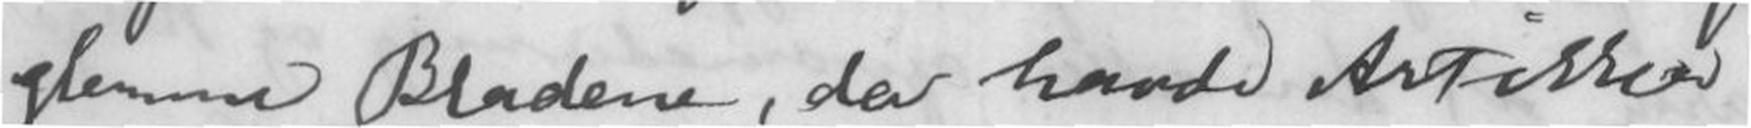glemme Bladene, der havde Artikler
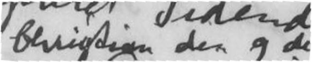Christian den 9de
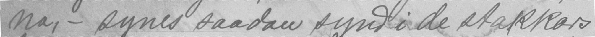na, - synes saadan synd i de stakkars
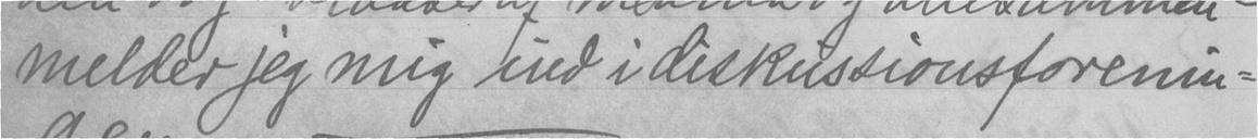melder jeg mig ind i diskussionsforenin-
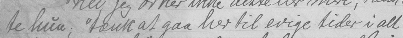te hun; "tænk at gaa her til evige tider i alt
